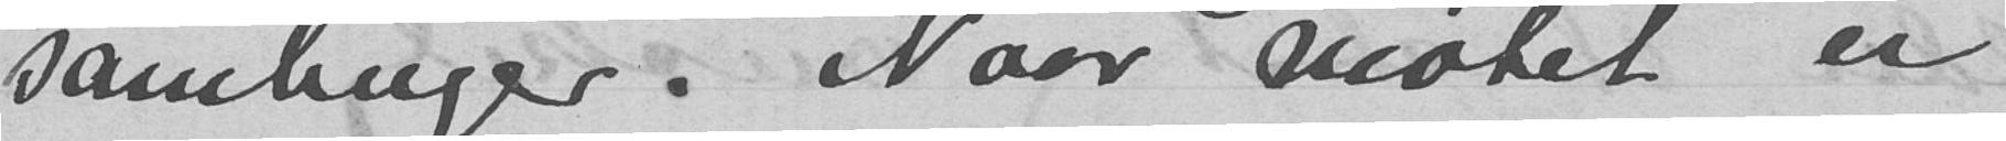samlinger. Naar møtet er
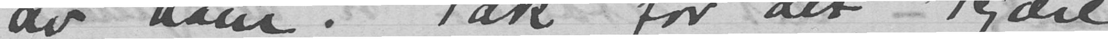av ham. Tak for det kjære
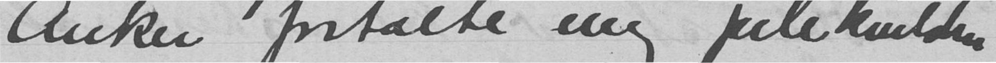Anker fortalte mig julekvelden
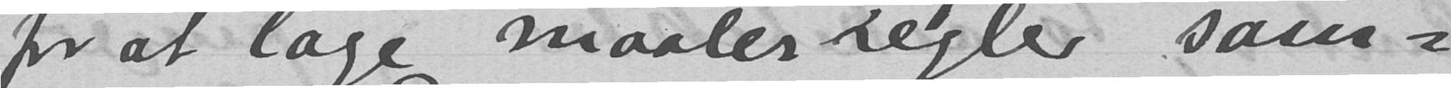for at lage maalerregler sam-
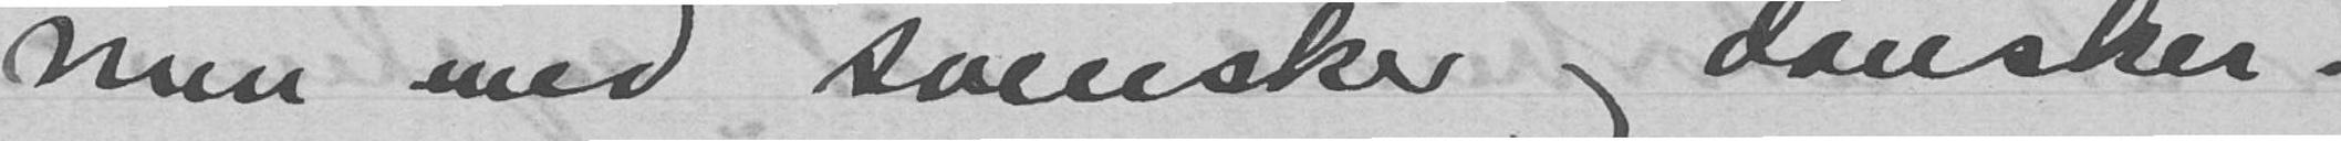men med svensker og dansker.
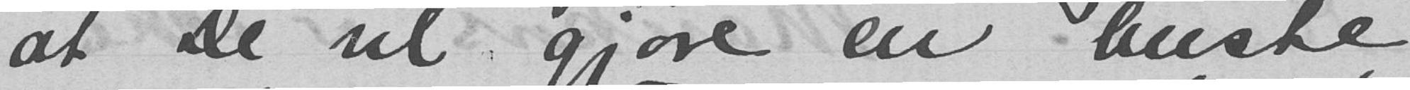at De vil gjøre en buste
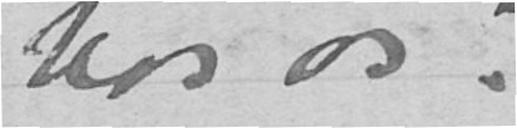hos os.
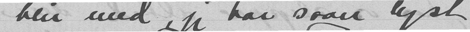Jeg blir med, jeg har saan lyst
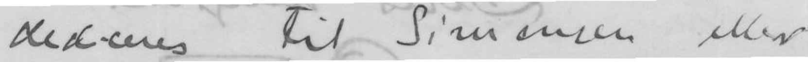dedseres til Simensen eller
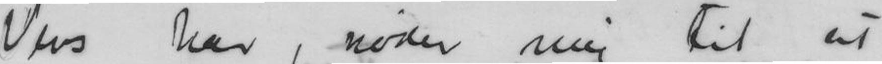Vers har, røder mig til at
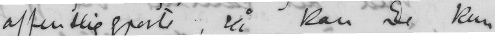offentliggjort, så kan De kun
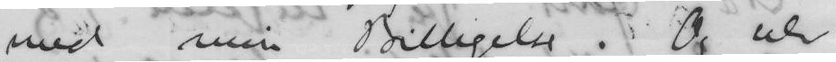med min Billigelse. Og selv
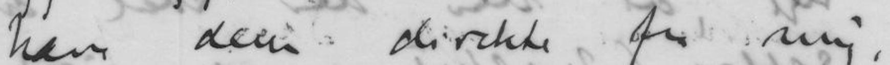have dem direkte fra mig,
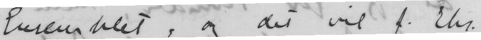Ensemblet, og det vil f. Eks.
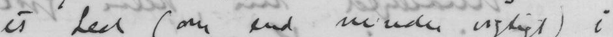et Led (Om end mindre vigtigt) i
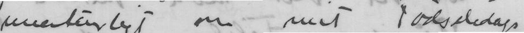unaturligt om mit Fødselsdagsbrev
